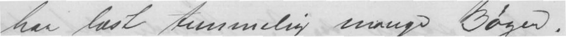har læst temmelig mange Bøger.
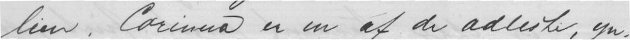lien. Corinna er en af de adleste, yn-
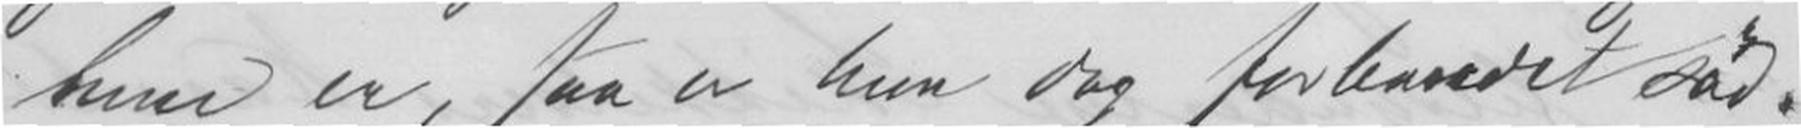hun er, saa er hun dog forbandet sød.
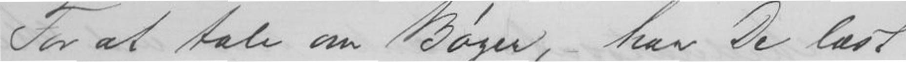For at tale om Bøger, - har De læst
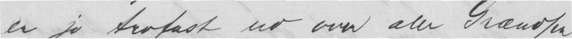er jo trofast ud over alle Grændser,
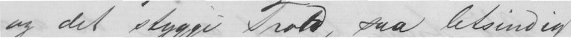og det stygge Trold, saa letsindig
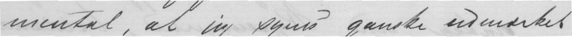mental, at jeg synes ganske udmærket
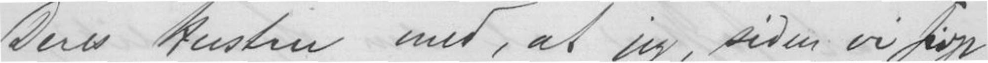Deres Hustru med, at jeg, siden vi sidst
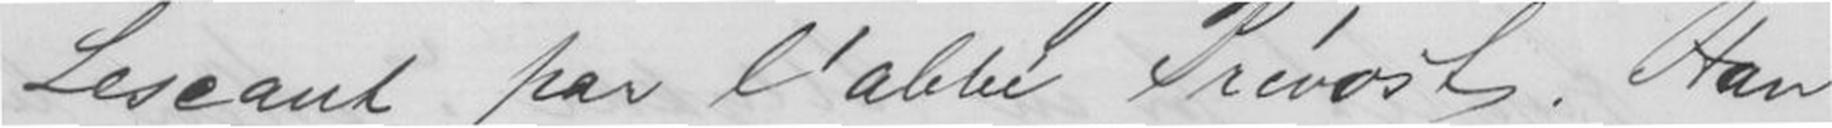Lescant par l'abbé Prévost. Han
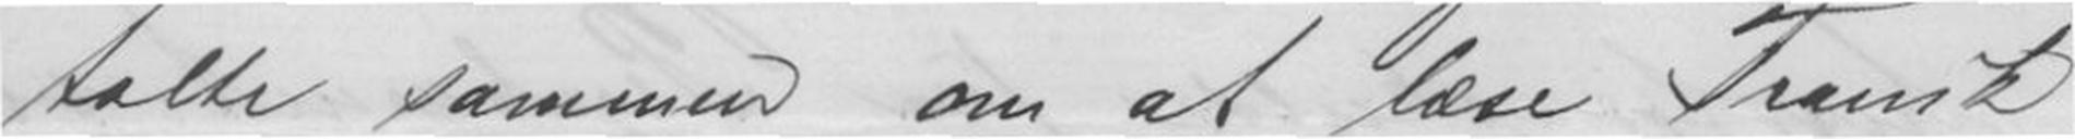talte sammen om at læse Fransk,
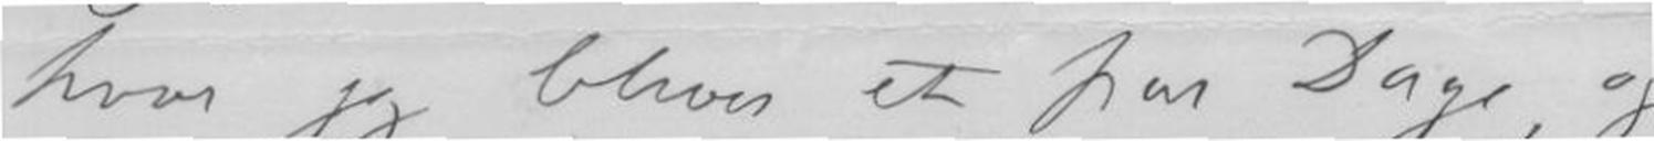hvor jeg bliver et par Dage, og
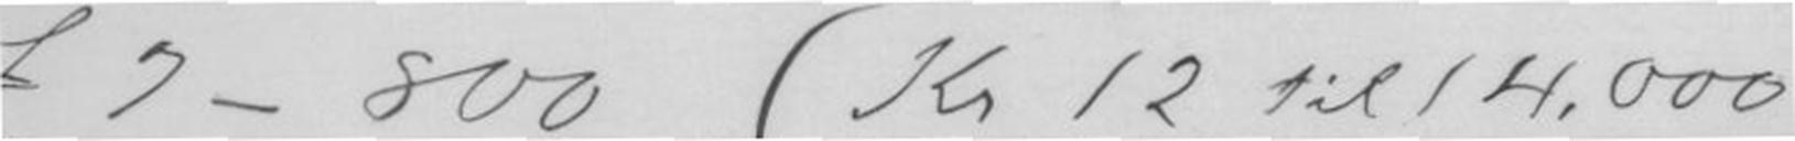£7-800 (Kr 12 til 14.000)
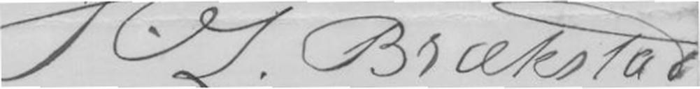H.L. Brækstad
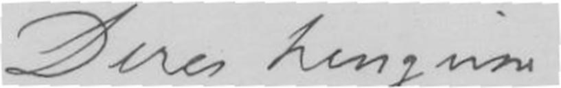Deres hengivne
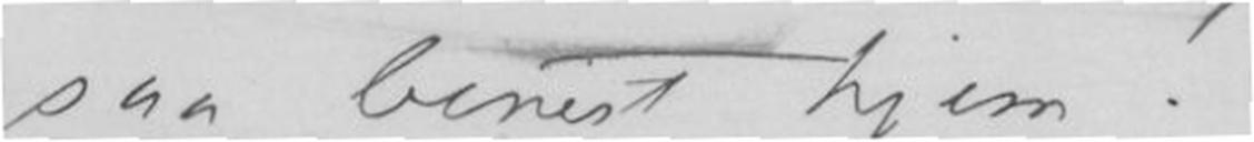saa benest hjem!
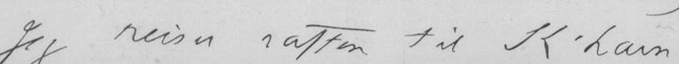Jeg reiser iaften til K'havn,
